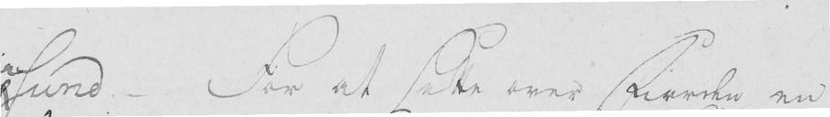sund. - For at sette over Fiorden en
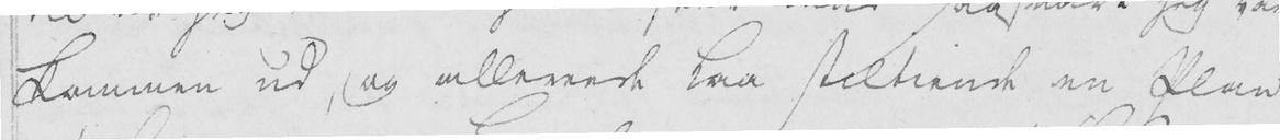kommen ud, og allereede laa stiltiende en Plan
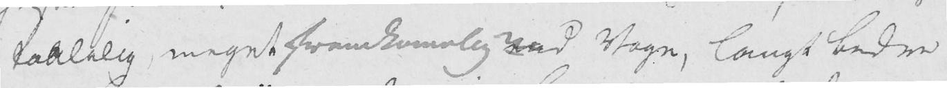taalelig, meget fremkomelig med Vogn, langt bedre
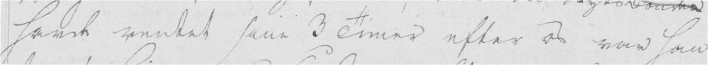havde ventet sine 3 Timer efter os var han
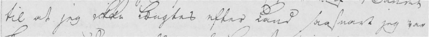til at jeg ikke længtes efter Land saasnart jeg var
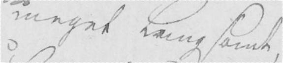meget langsomt,
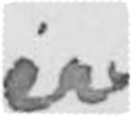er
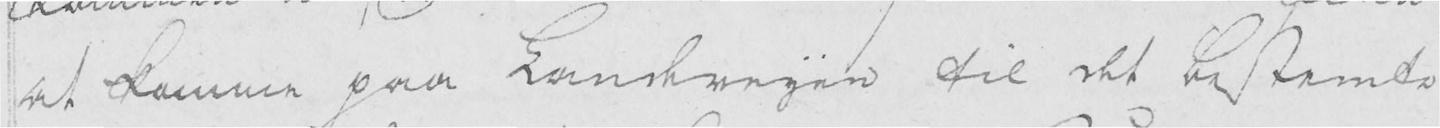at komme paa Landeveyen til det bestemte
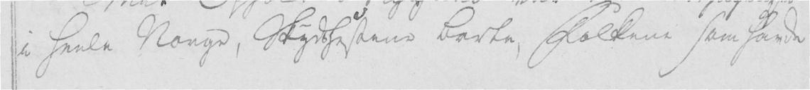i heele Norge, Skydshestene borte, Folkene som havde
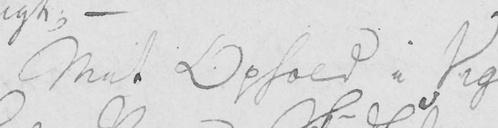Mit Ophold i Vig
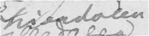skiendalen
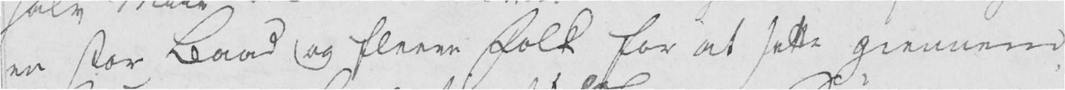en stor Baad og fleere folk for at sette giennem
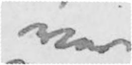var
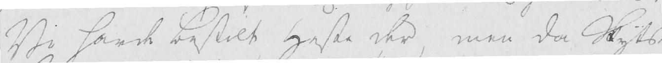Vi havde bestilt Heste der, men da Skyts
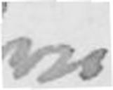vi
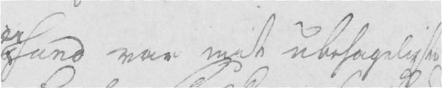sund var mit ubehageligste
